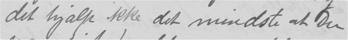det hjalp ikke det mindste at Du
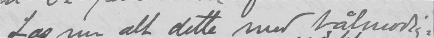Læs nu alt dette med tålmodig-
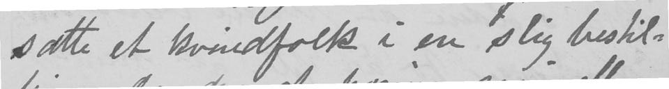sætte et kvindfolk i en slig bestil-
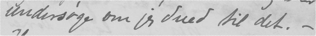undersøge om jeg dued til det. -
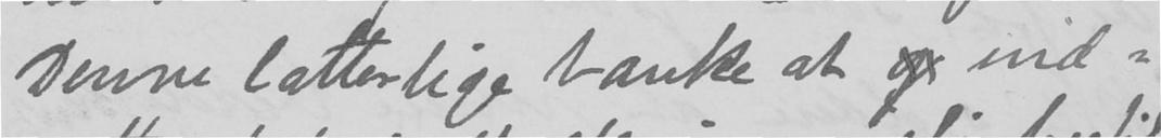Denne latterlige tanke at op ind-
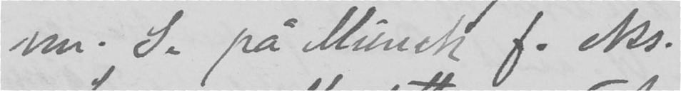nu. Se på Munch f.eks.
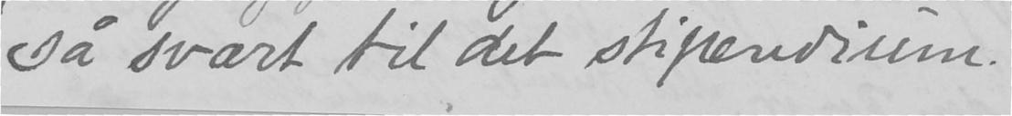så svært til det stipendium.
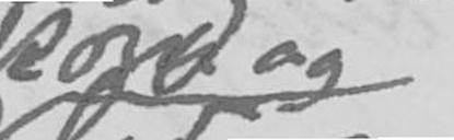kom og
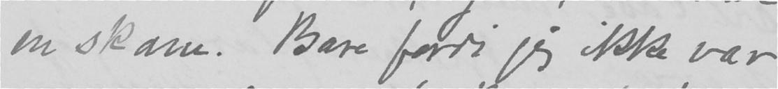en skam. Bare fordi jeg ikke var
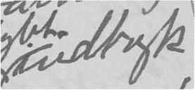indtryk
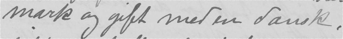mark og gift med en dansk,
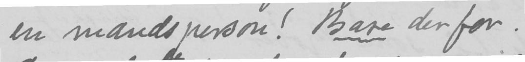en mandsperson! Bare derfor.
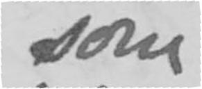som
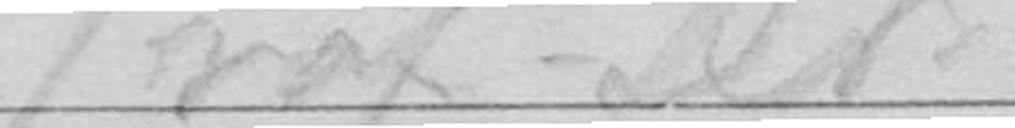Prof. Dr.
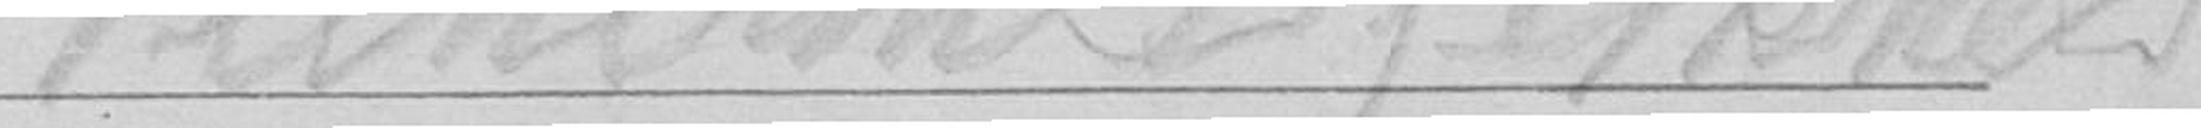Vilhelm Bjerknes
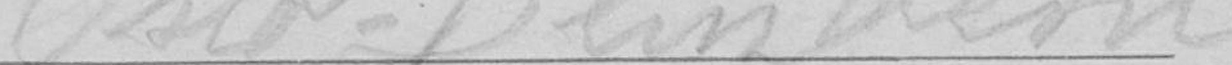Oslo-Blindern
